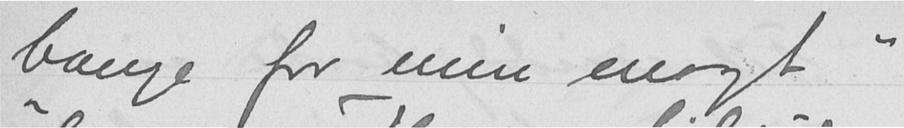"bange for min magt"
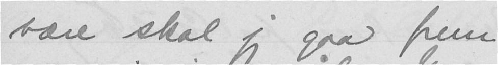bære skal jeg gaa frem
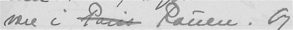være i Paris Rouen. Og
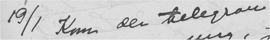19/1 Kom der telegram
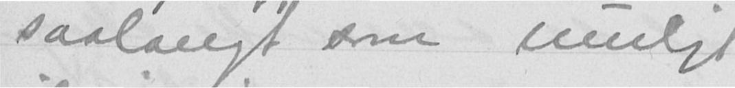saalangt som muligt
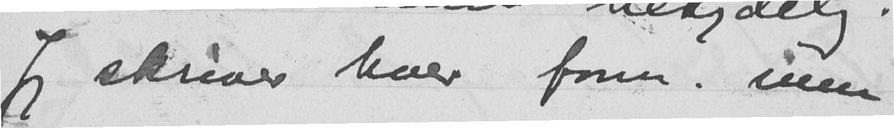Jeg skriver hver form. men
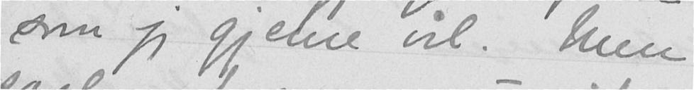som jeg gjerne vil. Men
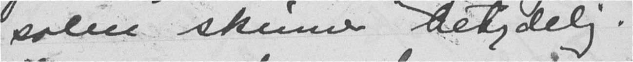solen skinner betydelig.
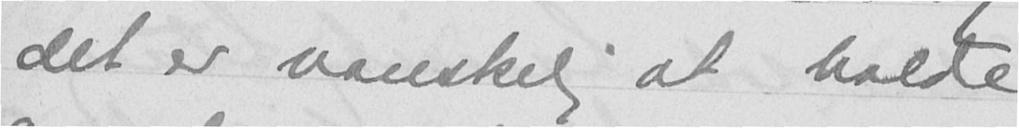det er vanskelig at holde
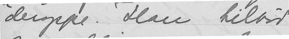deroppe. Han tilbød
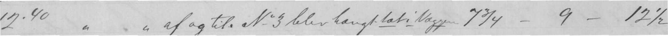" 12.40" " af og til. N.3 blir hængt tæt i Væggen 73/4 - 9 - 12½
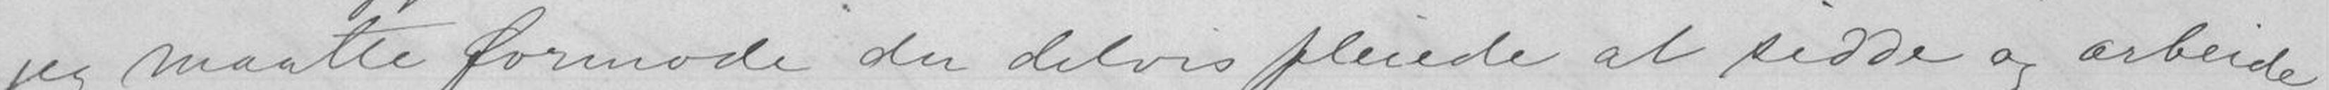jeg maatte formode du delvis pleiede at sidde og arbeide
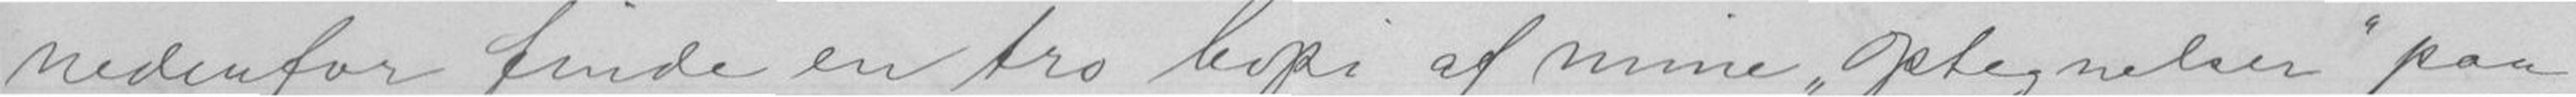nedenfor finde en tro Copi af mine Optegnelser paa
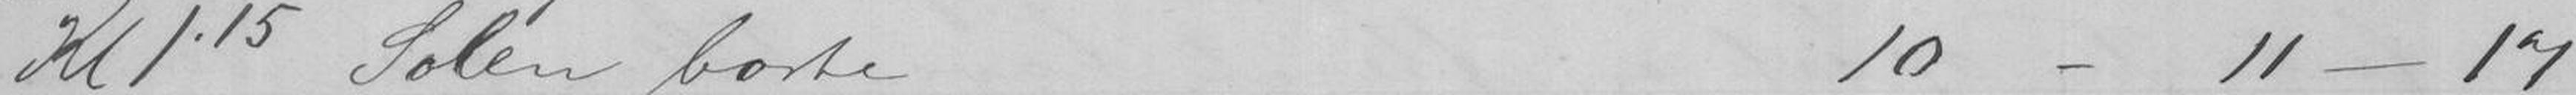Kl 1.15 Solen borte10 - 11 - 17
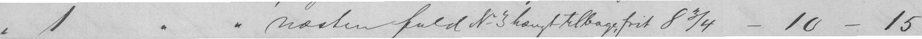" 1" " næsten fuld N.3 hængt tilbage, frit 83/4 - 10 - 15
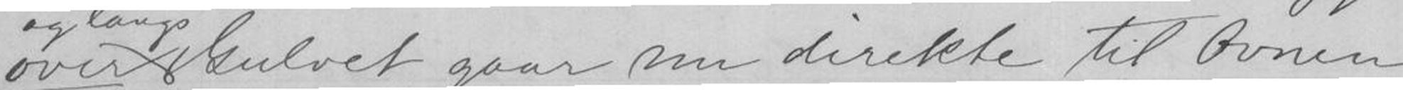over Gulvet gaar nu direkte til Ovnen
